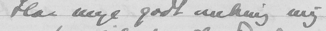Har mye godt omkring mig
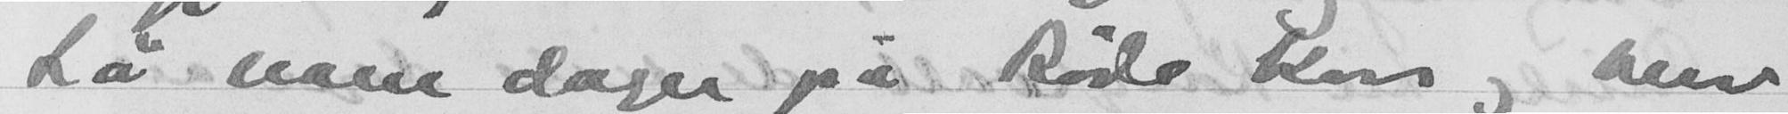Lå noen dager på Røde Kors og blev
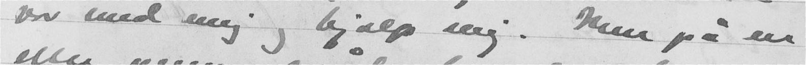var med mig og hjalp mig. Men på en
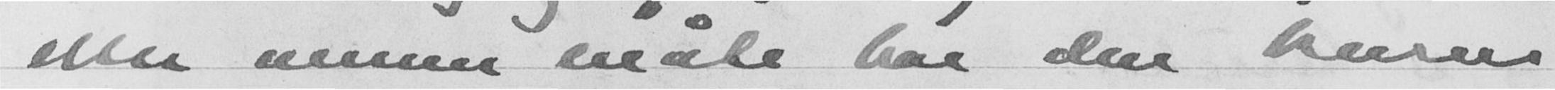eller annen måte har den busen
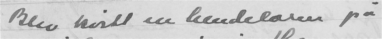Blev kvitt en hendeløre på
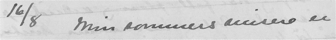16/8 Min sommers misere er
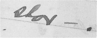stor - .
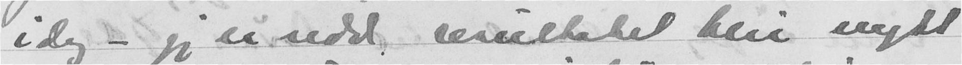i dag - jeg er redd resultatet blir nytt
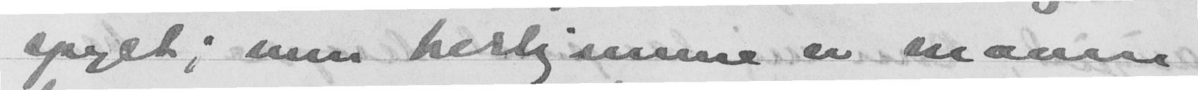spylt; men herhjemme er maven
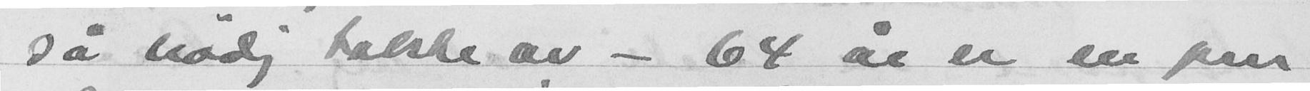så nødig takke av - 64 år er en pen
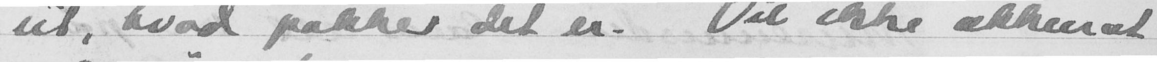ut, hvad pokker det er. Vil ikke akkurat
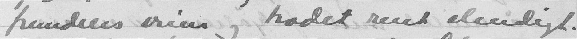fremdeles vrien og hodet rent elendigt.
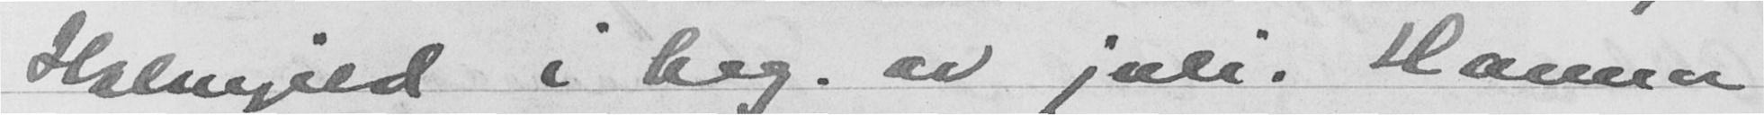Holmgild i beg. av juli. Hanna
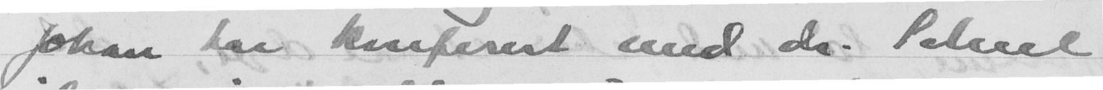Johan har konferert med dr. Schul
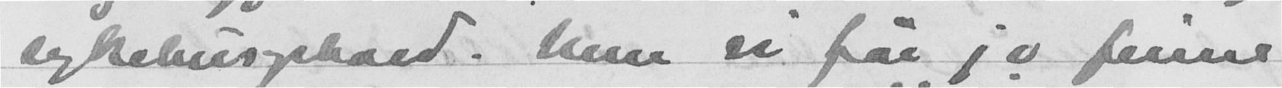sykehusophold. Men vi får jo finne
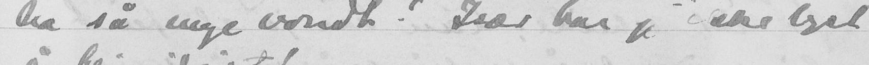ha så mye vondt. Frær har jeg ikke lyst
